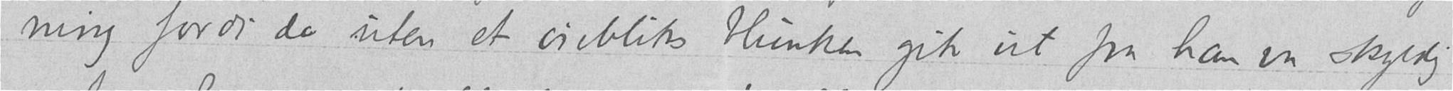ning fordi de uten et øiebliks blunken gik ut fra han var skyldig
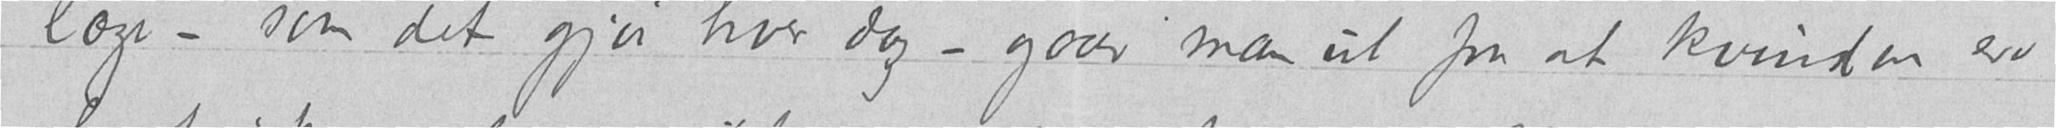læge – som det gjør hver dag – gaar man ut fra at kvinden er
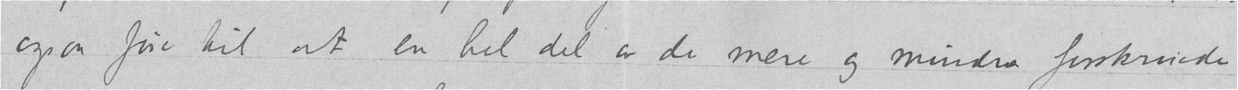ogsaa føre til at en hel del av de mere og mindre forskruede
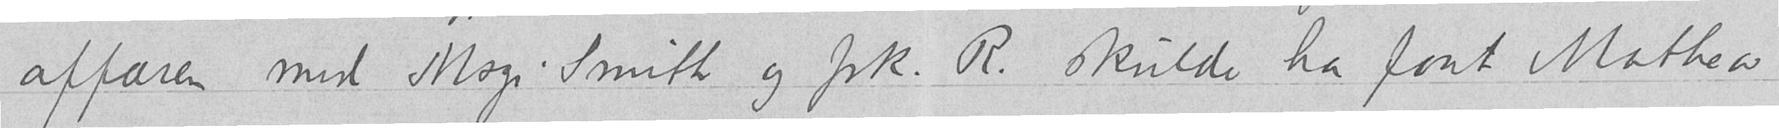affæren med Msgr. Smith og frk. R. skulde ha faat Mathea
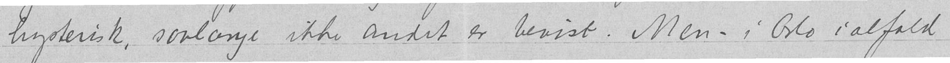hysterisk, saalænge ikke andet er bevist. Men – i Oslo ialfald
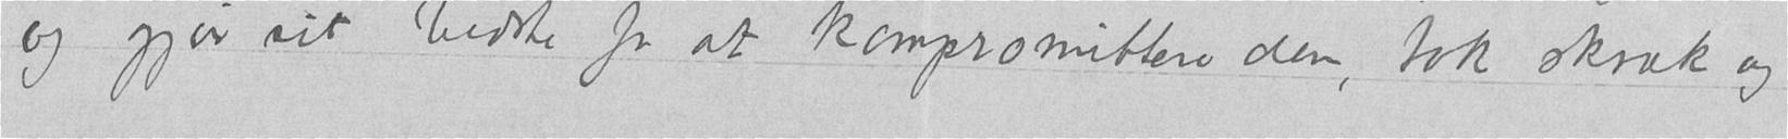og gjør sit bedste for at kompromittere dem, tok skræk og
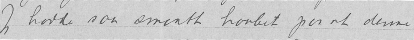Jeg hadde saa smaatt haabet paa at denne
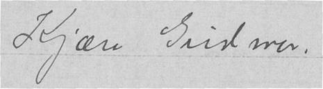Kjære Gudrun,
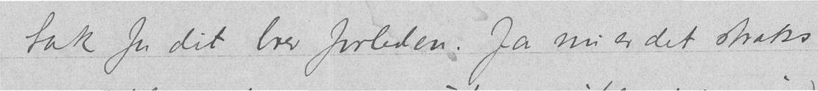tak for dit brev forleden. Ja nu er det straks
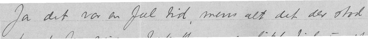Ja det var en fæl tid, mens alt det der stod
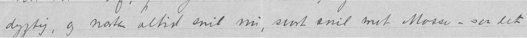dygtig, og næsten altid snil nu, svært snil mot Mosse – saa det
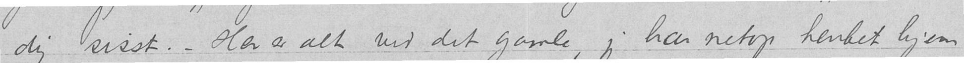dig sisst. – Her er alt ved det gamle, jeg har netop hentet hjem
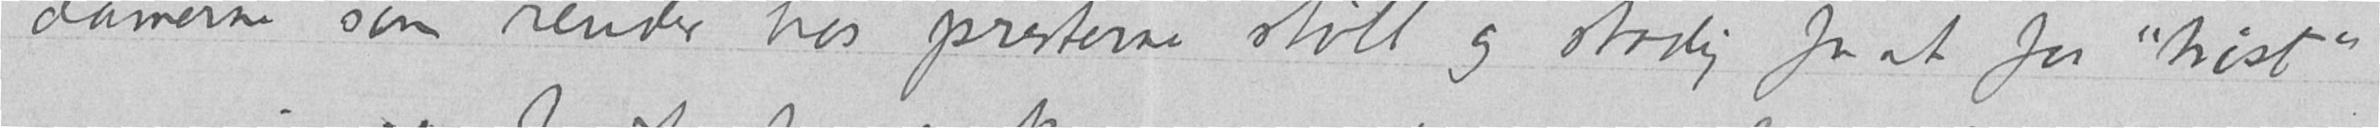damerne som render hos presterne støtt og stadig for at faa "trøst"
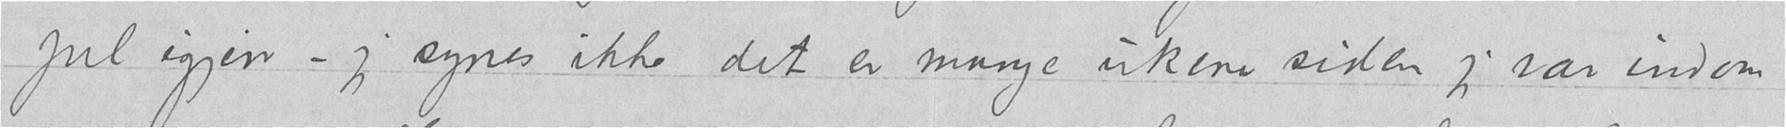jul igjen – jeg synes ikke det er mange ukene siden jeg var indom
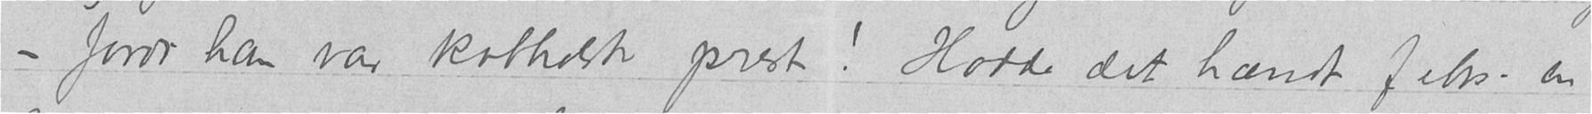– fordi han var katholsk prest! Hadde det hændt f eks. en
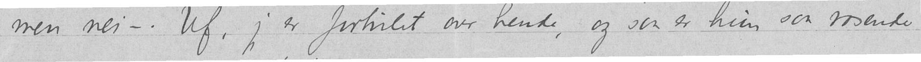men nei –. Uf, jeg er fortvilet over hende, og saa er hun saa rasende
